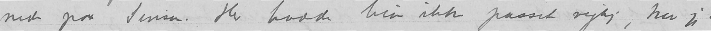nede paa Sinsen. Her hadde hun ikke passet rigtig, tror jeg.
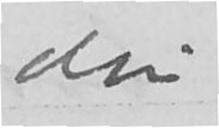din
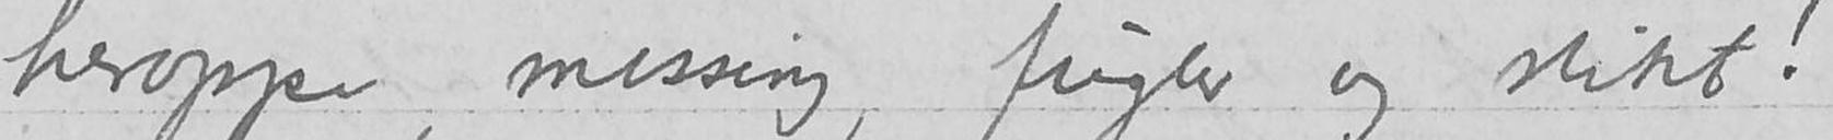heroppe, messing, fugler og slikt!
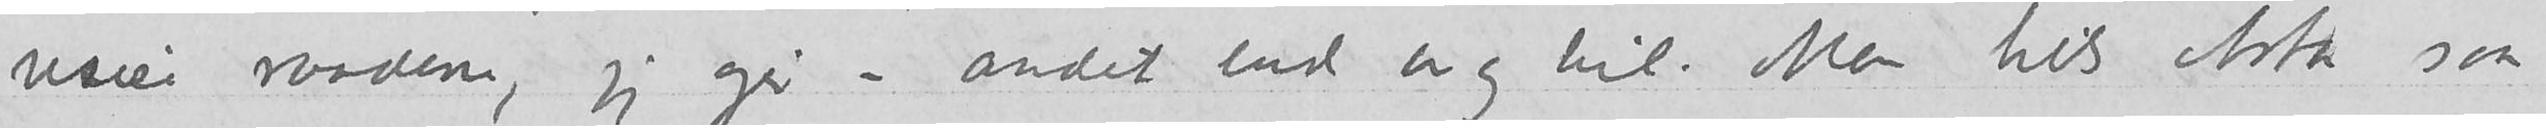vise raadene, jeg gir – andet end av og til. Men hils Asta
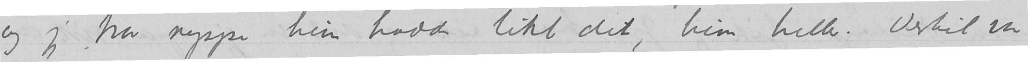og jeg tror neppe hun hadde likt det, hun heller. Dertil var
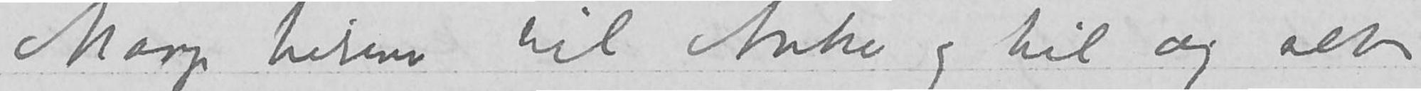Mange hilsener til Anker og til dig selv
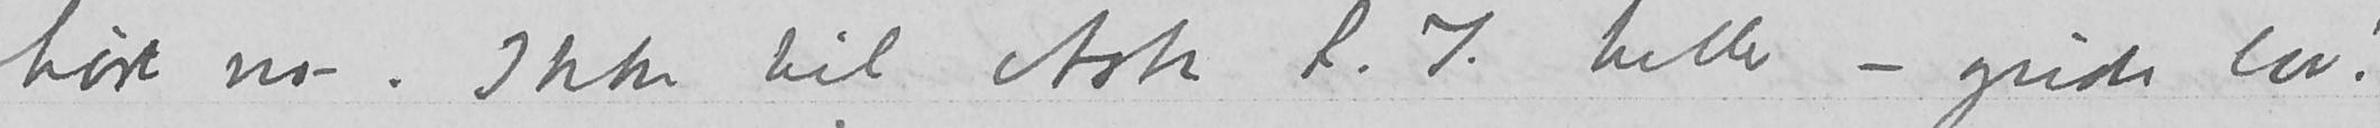Ikke til Asta L.I. heller – guds lov!
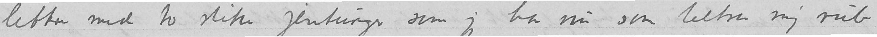lettere med to slike jentunger som jeg har nu som betror mig rub
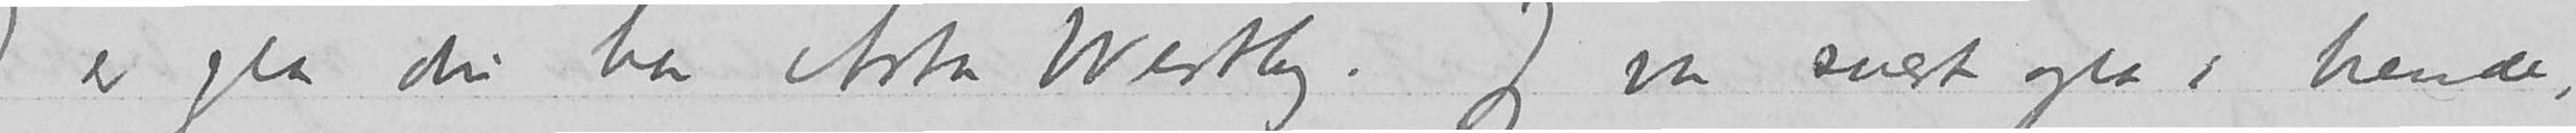Jeg er gla du har Asta Westby. Jeg var svært gla i hende,
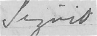Sigrid
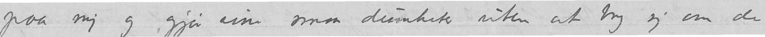paa mig og gjør sine smaa dumheter uten at bry sig om de
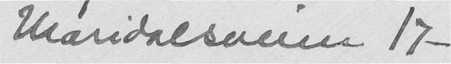Maridalsveien 17-
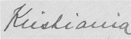Kristiania
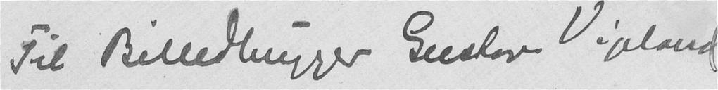Til Billedhugger Gustav Vigeland
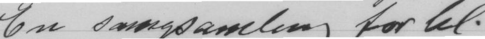En songsamling for bl.
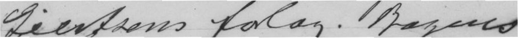Giertsens forlag. Bogens
